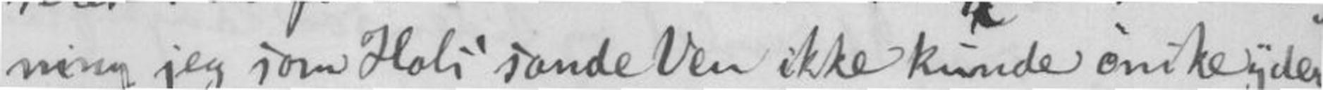ning jeg som Hals sande Ven ikke kunde ønske yder-
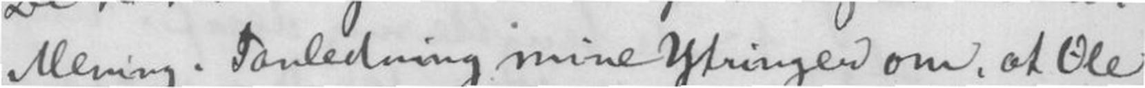Mening. Ianledning mine Ytringer om, at Ole
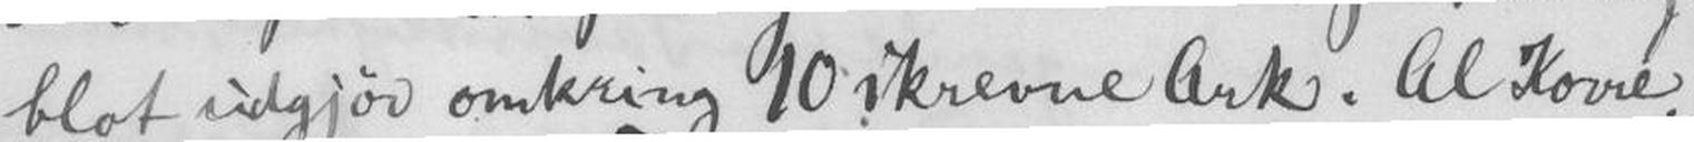blot udgjør omkring 10 skrevne Ark. Al Korre-
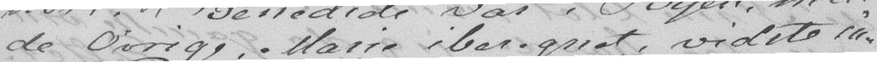de Øvrige, Marie iberegnet, vidste in-
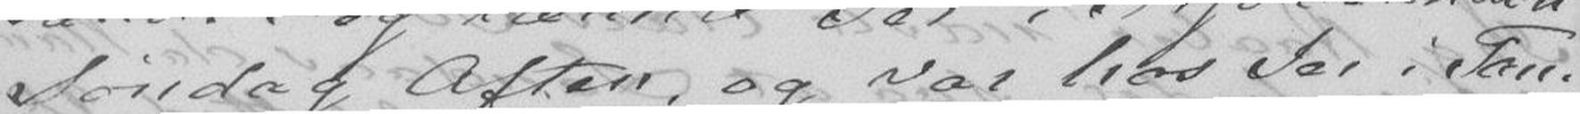Søndag Aften, og var hos Jer i Tan-
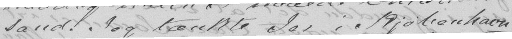sand. Jeg tænkte Jer i Kjøbenhavn
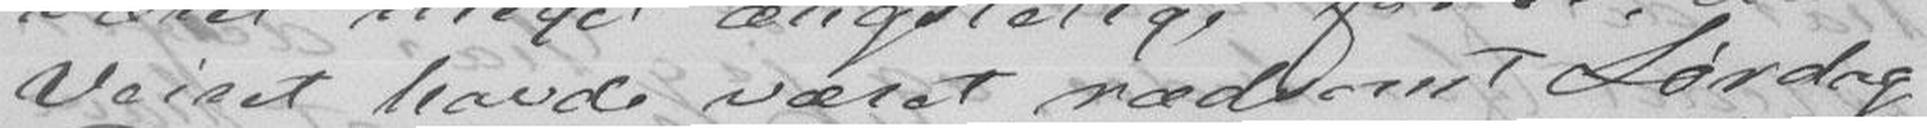Veiret havde været rædsomt Lørdag
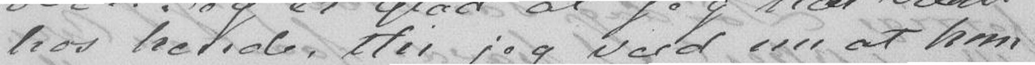hos hende, thi jeg veed nu at hun
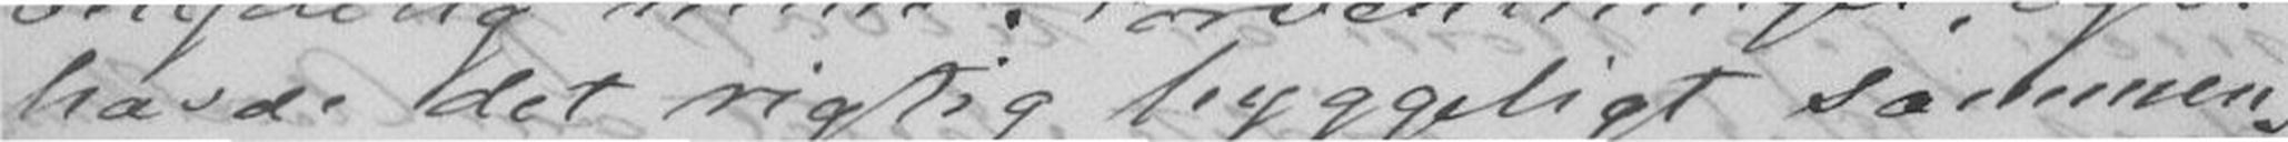havde det rigtig hyggeligt sammen.
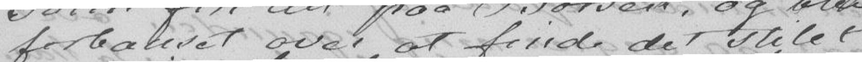forbauset over at finde det stilet
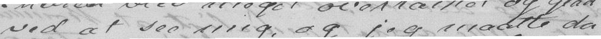ved at see mig, og jeg maatte da
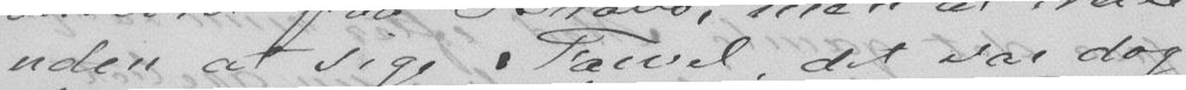uden at sige Farvel, det var dog
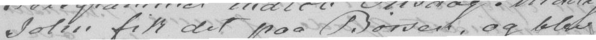John fik det paa Børsen, og blev
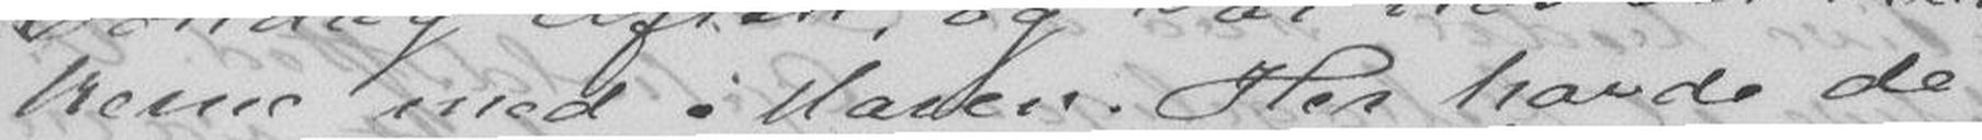kerne med Maren. Her havde de
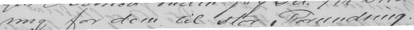mig for den til stor Forundring.
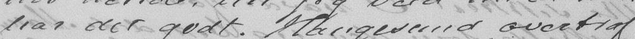har det godt. Haugesund overtraf
